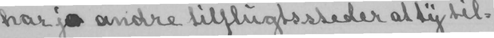har jo andre tilflugtssteder at ty til-
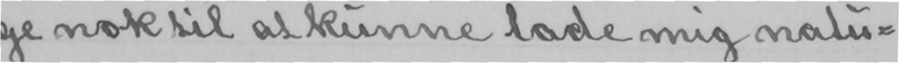ge nok til at kunne lade mig natu-
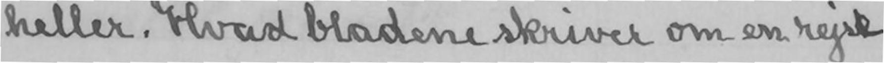heller. Hvad bladene skriver om en rejse
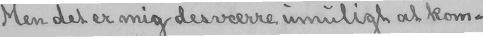Men det er mig desværre umuligt at kom-
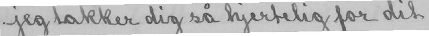jeg takker dig så hjertelig for dit
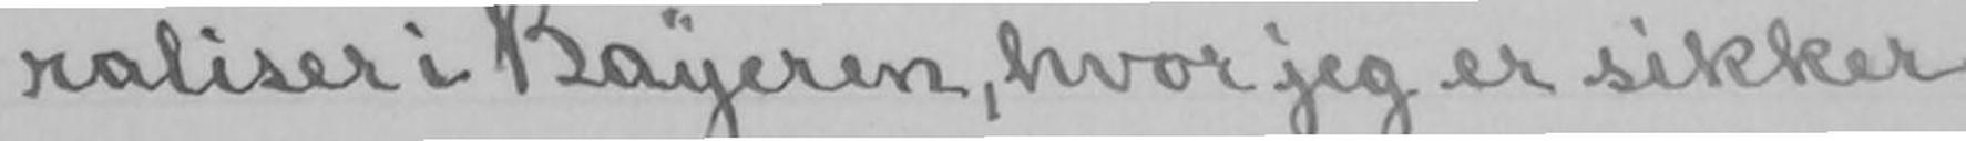raliser i Bayeren, hvor jeg er sikker
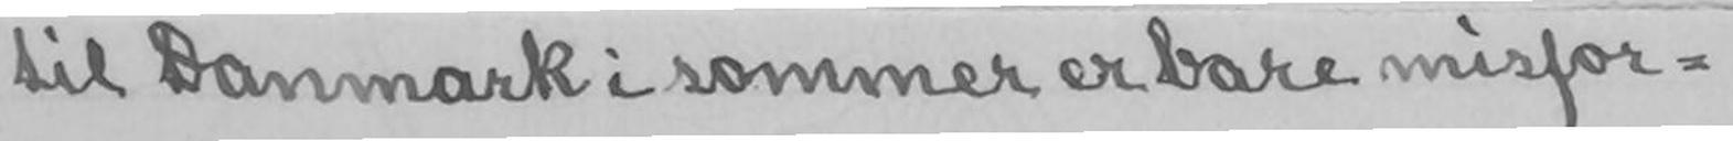til Danmark i sommer er bare misfor-
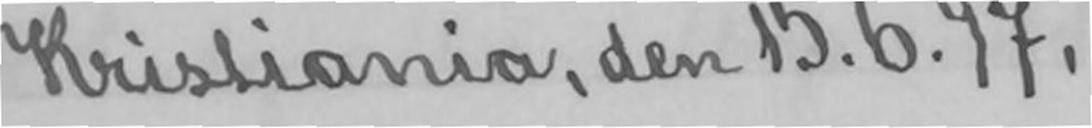Kristiania, den 15.6.97.
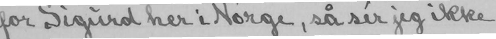for Sigurd her i Norge, så sér jeg ikke
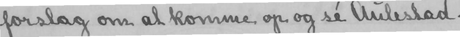forslag om at komme op og sé Aulestad.
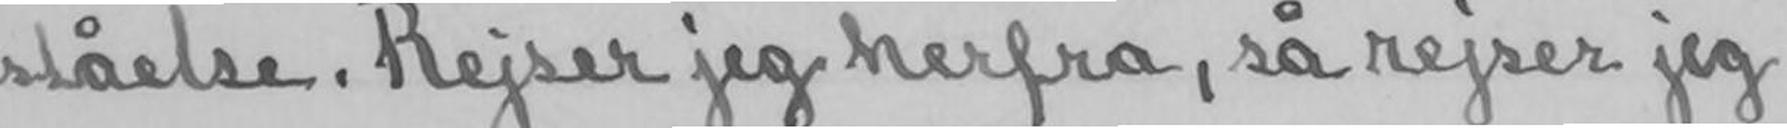ståelse. Rejser jeg herfra, så rejser jeg
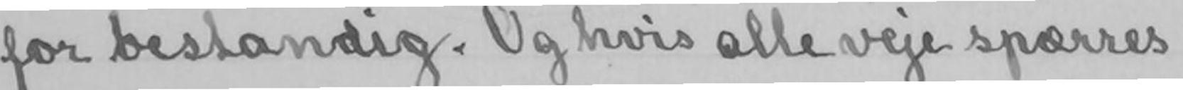for bestandig. Og hvis alle veje spærres
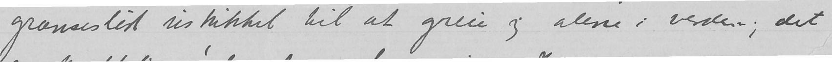grænsesløst uskikket til at greie seg alene i verden; det
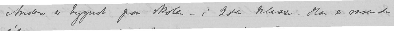Anders er begyndt paa skolen – i 2de klasse.  Han er rasende
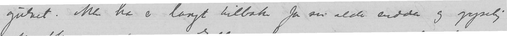gulvet. Men han er langt tilbake for sin alder endda og gyselig
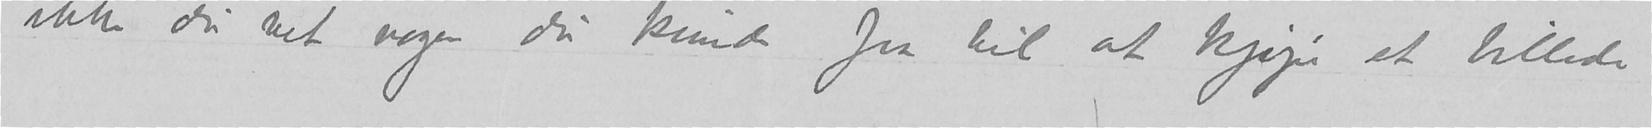ikke du vet nogen du kunde faa til at kjøpe et billede
